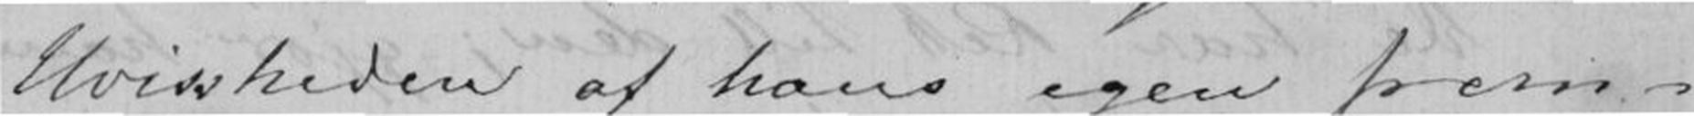Uvissheden af hans egen frem-
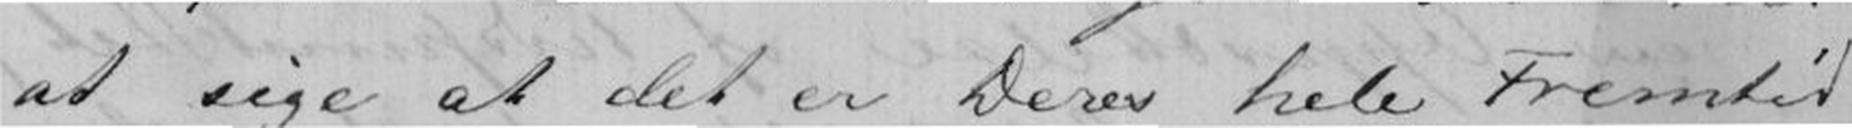at sige at det er Deres Fremtid
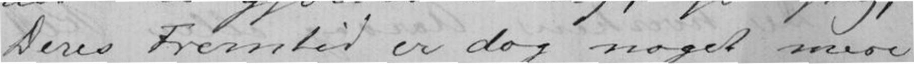Deres Fremtid er dog noget mere
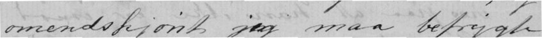omendskjønt jeg maa befrygte
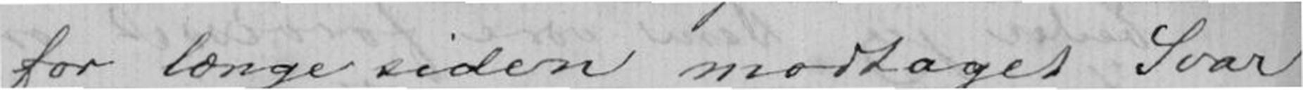for længe siden modtaget Svar
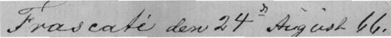Frascati den 24de August 66.
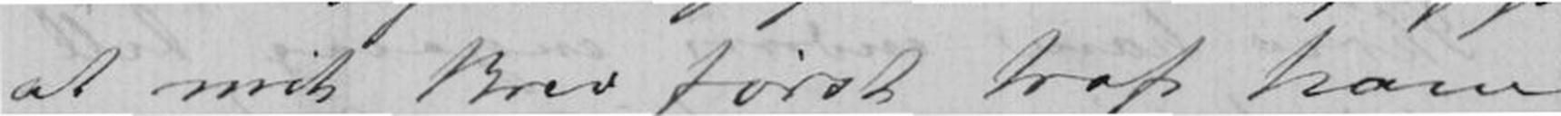at mit Brev først traf ham
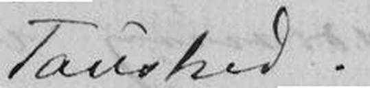Taushed.
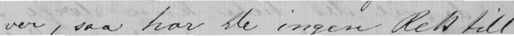ver, saa har De ingen Rett till
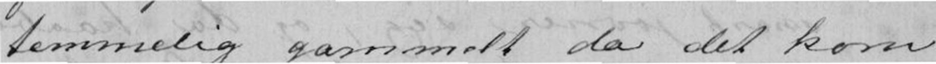temmelig gammelt da det kom
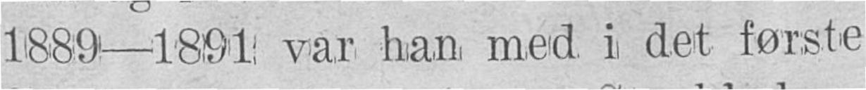1889-1891 var han med i det første
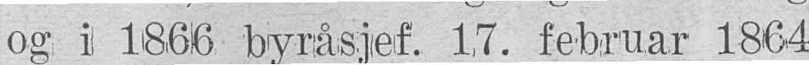og i 1866 byråsjeg. 18. februar 1864
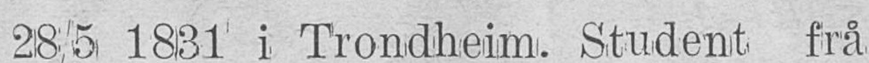28/5 1831 i Trondheim. Student frå
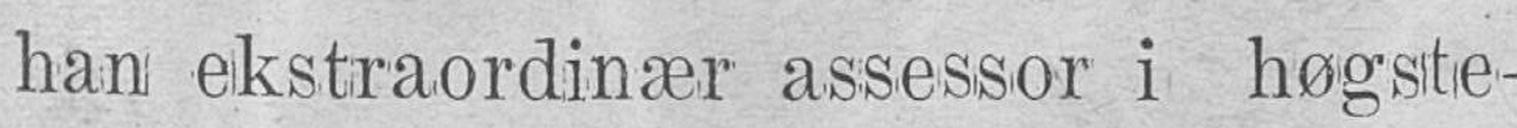han ekstraordinær assessor i høgste-
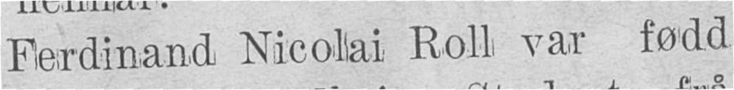Ferdinand Nicolai Roll var fødd
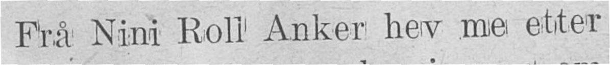Frå Nini Roll Anker hev me etter
